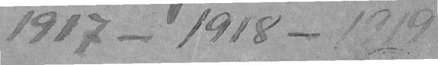1917 - 1918 - 1919
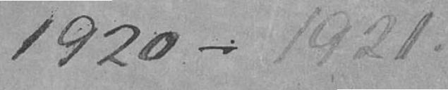1920 - 1921.
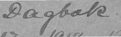Dagbok.
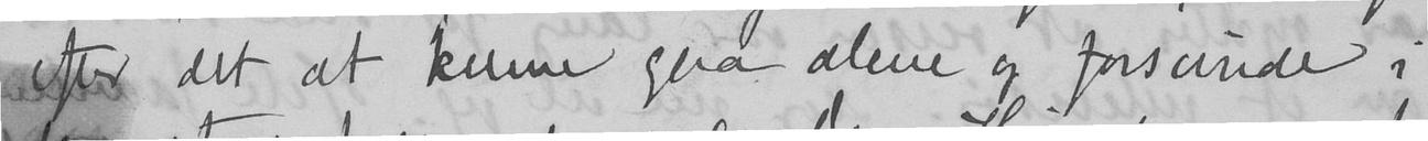efter det at kunne gaa alene og forsvinde i
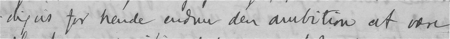digvis for hende endnu den ambition at være
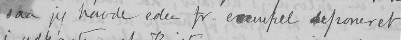saa jeg havde eder for exempel deponeret
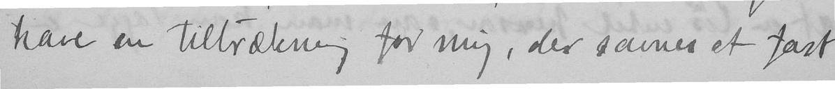have en tiltrækning for mig, der savnes et fast
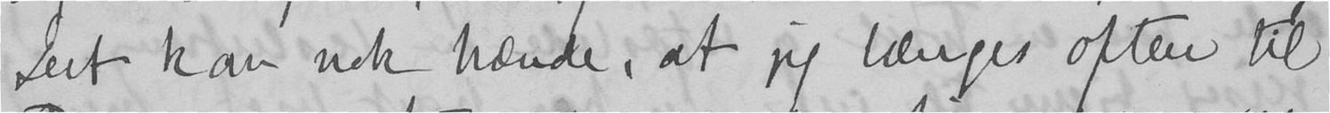Det kan nok hænde, at jeg længes oftere til
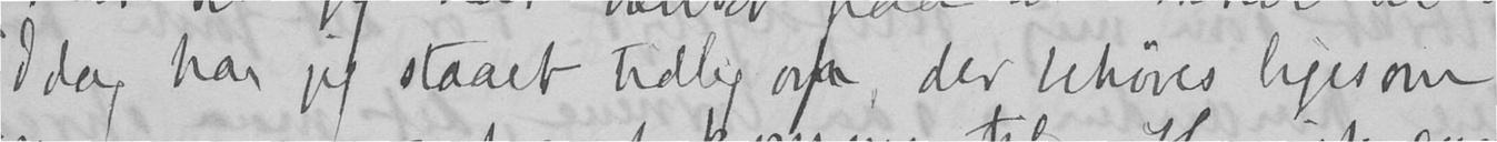Idag har jeg staaet tidlig op, der behøves ligesom
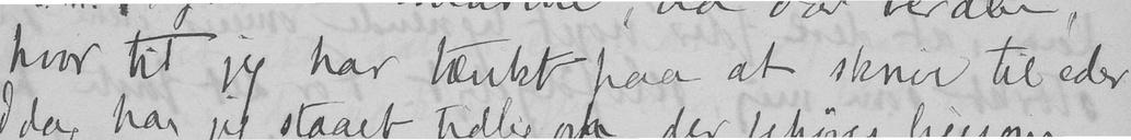hvor tit jeg har tænkt paa at skrive til eder
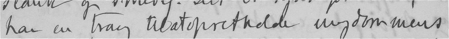har en trang til at opretholde ugdommens
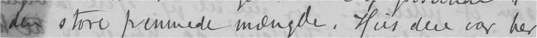den store fremmede mængde. Hvis dere var her,
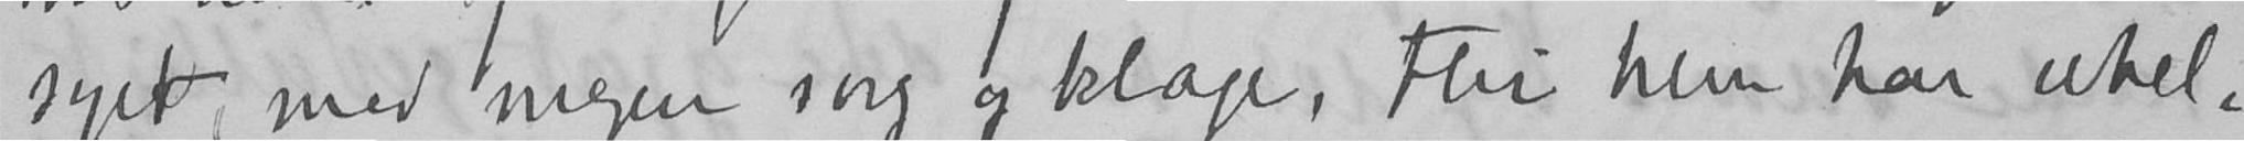syet med megen sorg og klage, thi hun har uhel-
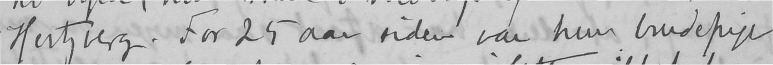Hertzberg. For 25 aar siden var hun brudepige
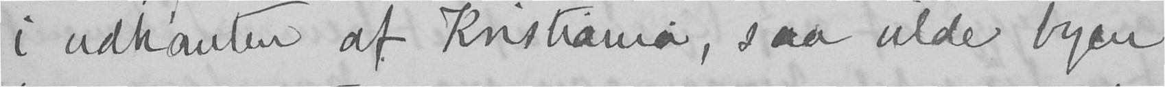i udkanten af Kristiania, saa vilde byen
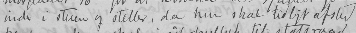inde i stuen og steller, da hun skal tidligt afsted
